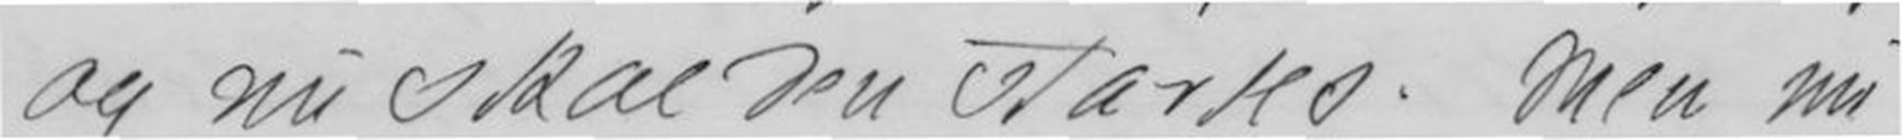og nu skal den startes. Men nu
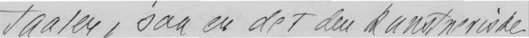Taaler, saa er det den kunstneriske
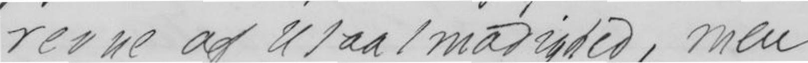revne af Utaalmodighed, men
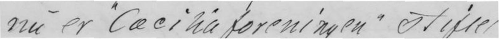nu er "Cæcihaforeningen" stiftet,
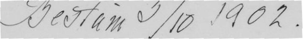Bestum 5/10 1902
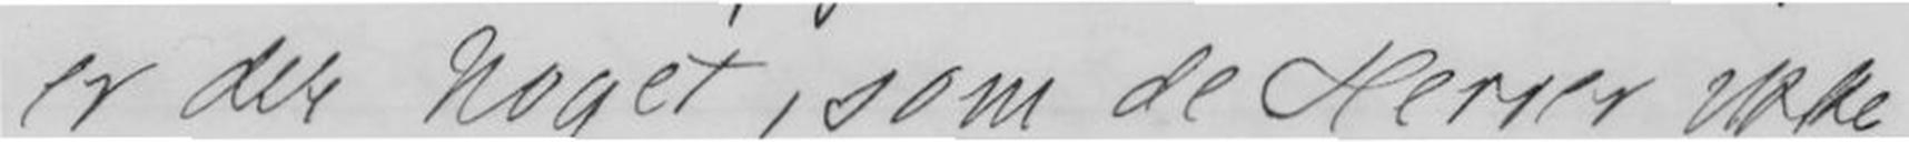er det noget, som de Herrer ikke
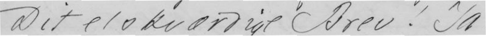Dit elskværdige Brev! Ja
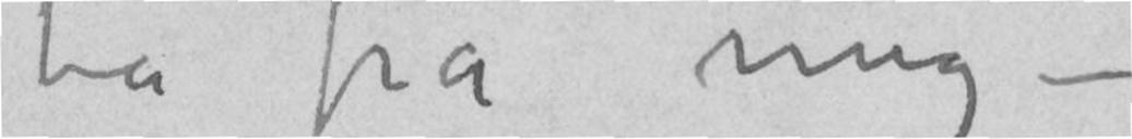ta fra mig –
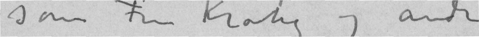som Fru Krohg og andre
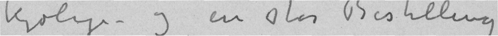kjølige – og en stor Bestilling
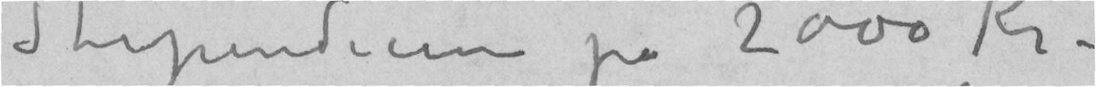Stipendium på 2000 Kr –
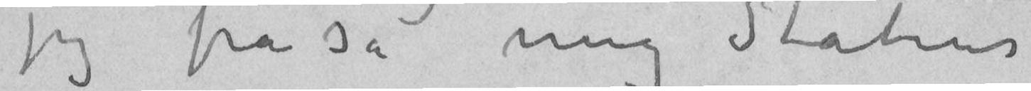jeg frasa mig Statens
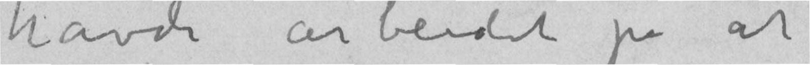havde arbeidet på at
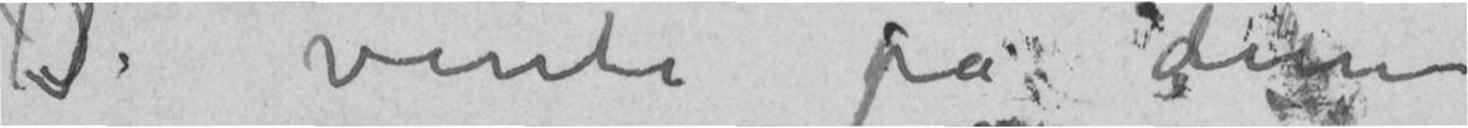I vente på denne
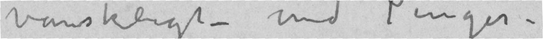vanskeligt – med Penger –
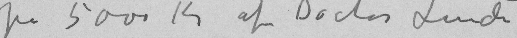på 5000 Kr af Doctor Linde
